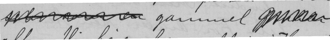gammel
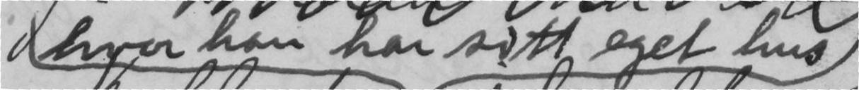hvor han har sitt eget hus
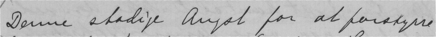Denne stadige Angst for at forstyrre
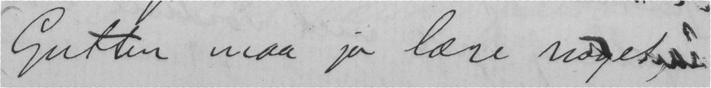Gutten maa jo lære noget,
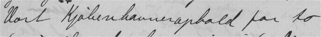Vort Kjøbenhavnerophold for to
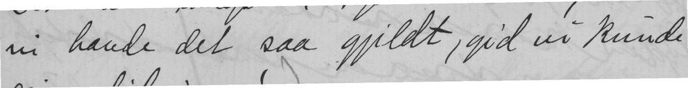vi havde det saa gjildt, gid vi kunde
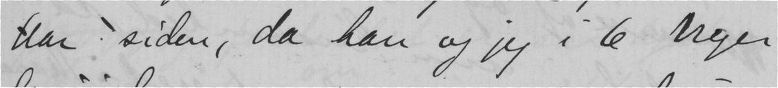aar siden, da han og jeg i 6 uger
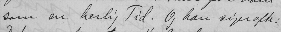som en herlig Tid. Og han siger ofte:
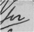for
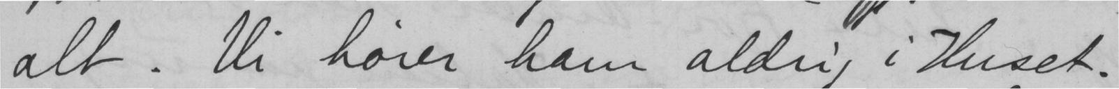alt. Vi hører ham aldrig i Huset.
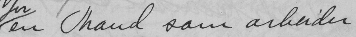en Mand som arbeider
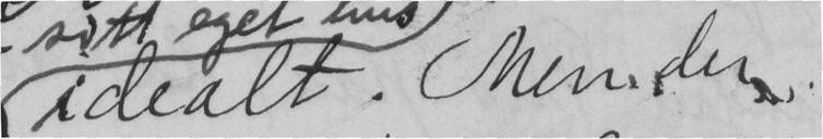idealt. Men der
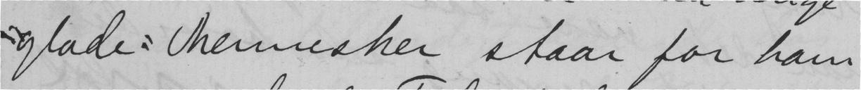"glade" mennesker staar for ham
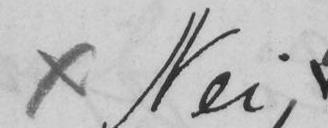x Nei,
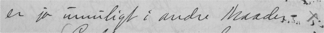er jo umuligt i andre Maader. x
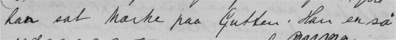har sat Mærke paa Gutten. Han er så
